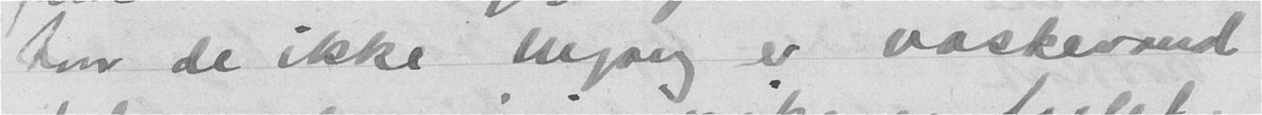hvor de ikke engang er vaskevand
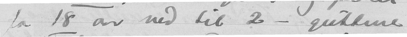paa 18 aar ned til 2 - guttene
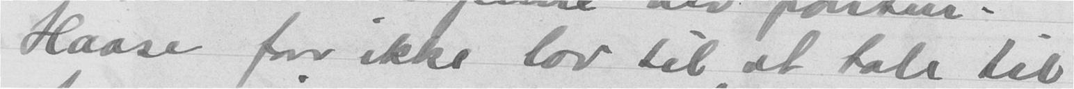Haase faar ikke lov til at tale til
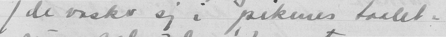(de vasker sig i pikenes toalet-
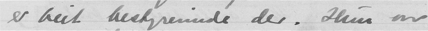er blit bestyrerinde der. Hun var
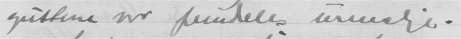guttene var fremdeles umulige.
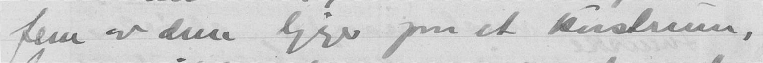fem av dem ligger paa et kvistrum,
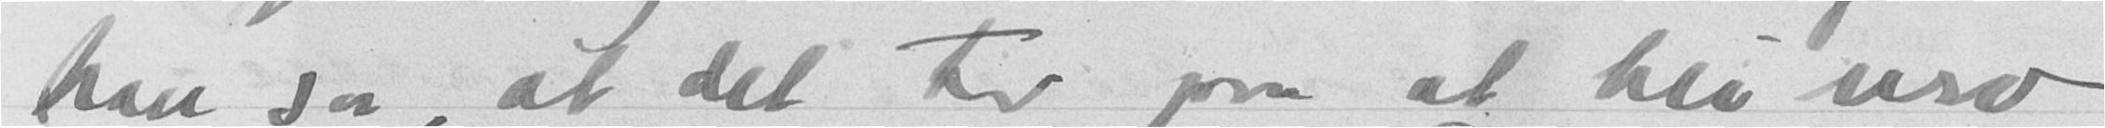han sa, at det tar paa at bli uro
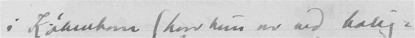i Kjøbenhavn (hvor hun var ved bolig-
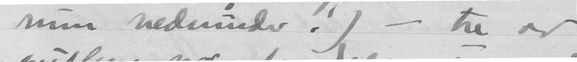rum nedenunder!) - tre av
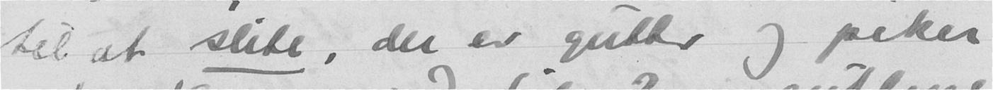til at slite, der er gutter og piker
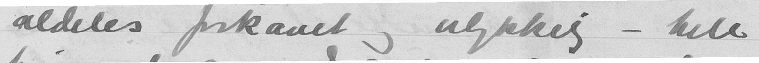aldeles forkavet og ulykkelig - hele
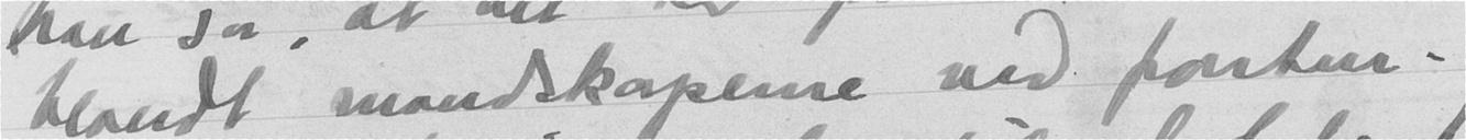blandt mandskapene ved fronten.
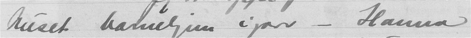huset barnehjem igaar - Hanna
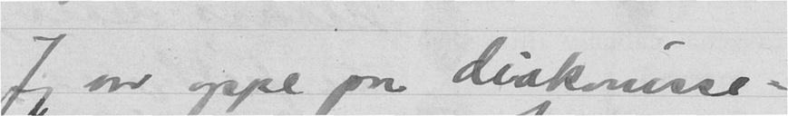Jeg var oppe paa diakonisse-
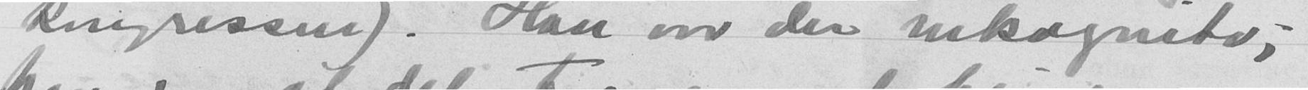kongressen). Han var der inkognito;
